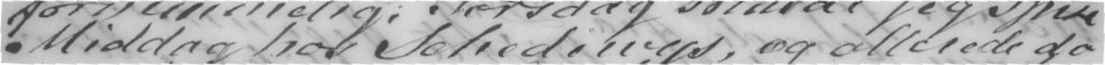Middag hos Schediwys, og allerede da
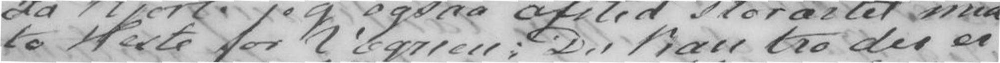to Heste for Vognen; Du kan tro der er
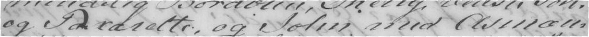og Paxarette, og John med Asman-
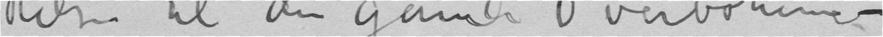Hilsen til den gamle Overbohem –
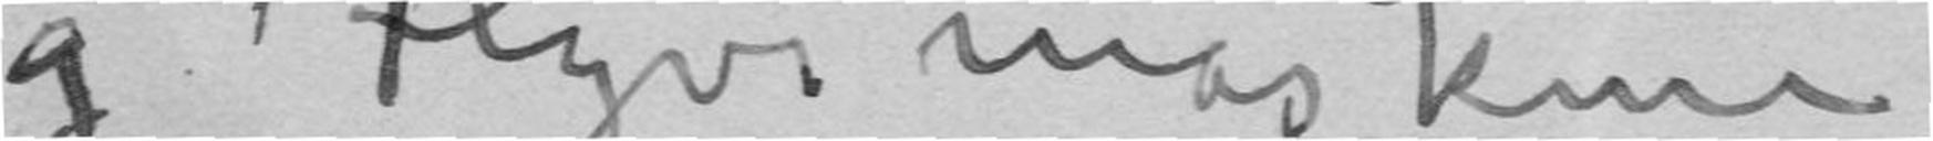og Flyvemaskine
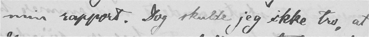min rapport. Dog skulde jeg ikke tro, at
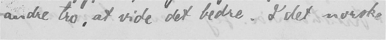andre tro, at vide det bedre. I det norske
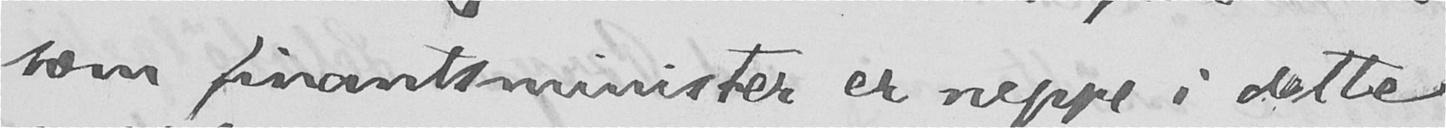som finantsminister er neppe i dette
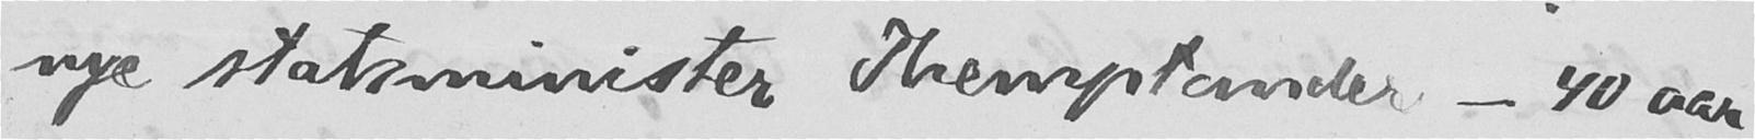nye statsminister Themptander - 40 aar
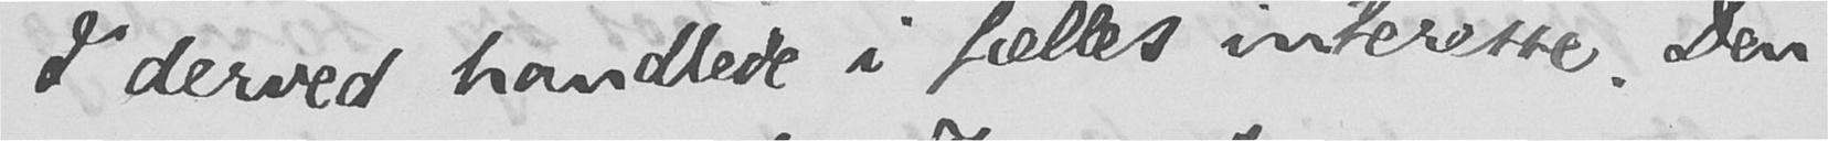I derved handlede i fælles interesse. Den
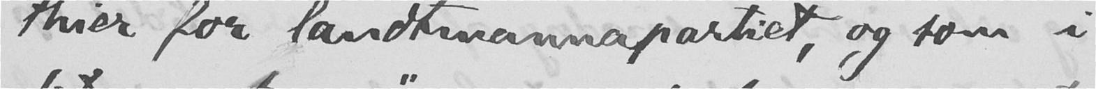thier for landtmannapartiet, og som i
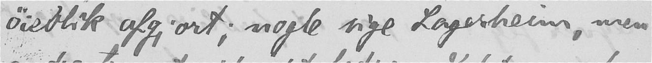øieblik afgjort; nogle sige Lagerheim, men
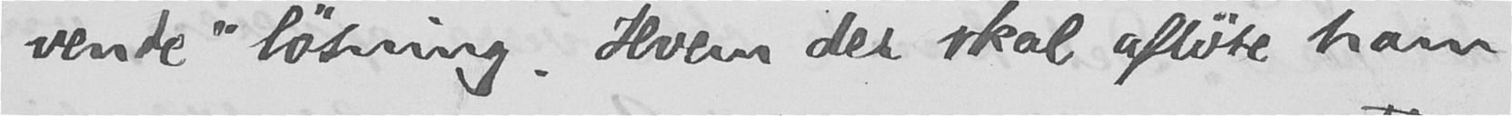vende" løsning. Hvem der skal afløse ham
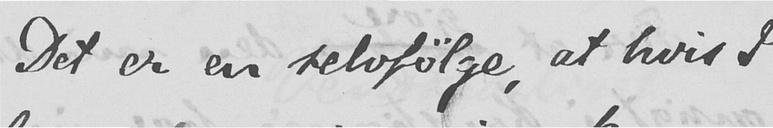Det er en selvfølge, at hvis I
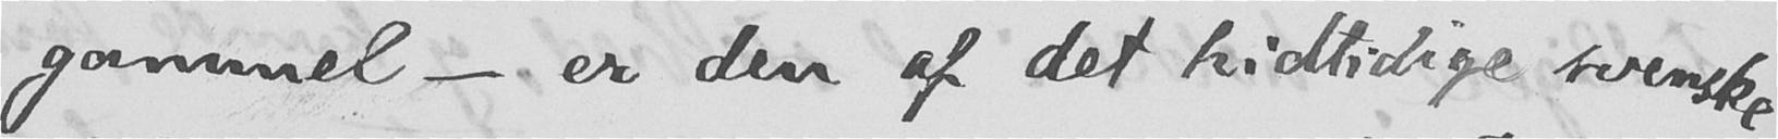gammel - er den af det hidtidige svenske
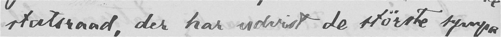statsraad, der har udvist de største sympa-
